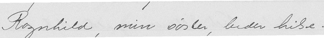Ragnhild, min søster, beder hilse.
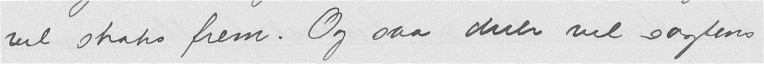vel straks frem. Og saa duer vel sagtens
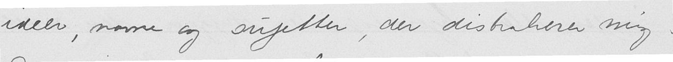ideer, navne og sujetter, der distraherer mig.
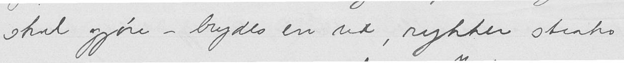skal gjøre - brydes en ud, rykker straks
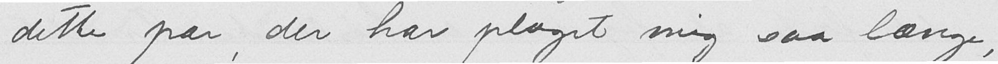dette par, der har plaget mig saa længe,
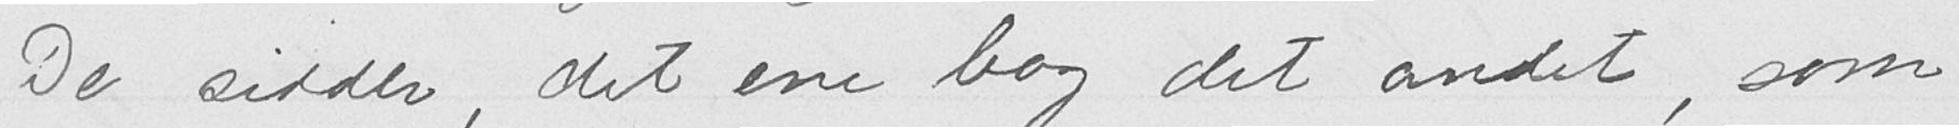De sidder, det ene bag det andet, som
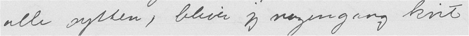alle sytten; bliver jeg nogengang kvit
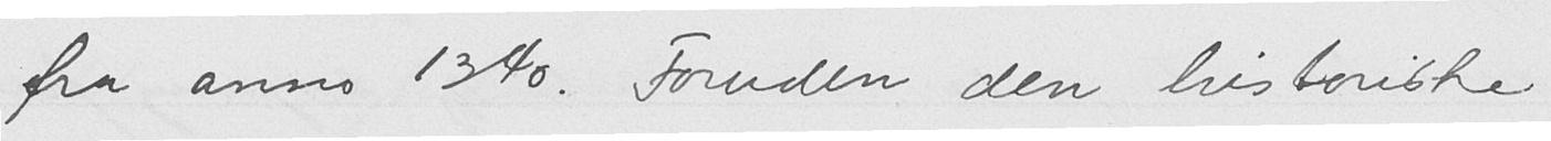fra anno 1340. Foruden den historiske
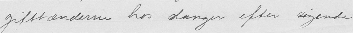gifttænderne hos slangen efter sigende
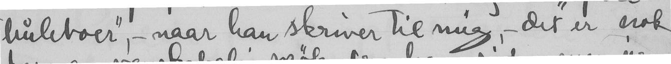huleboer", - naar han skriver til mig, - det er nok
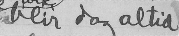blir dag altid
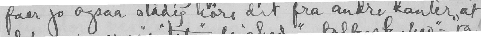faar jo ogsaa stadig høre det fra andre kanter, at
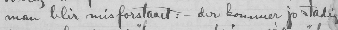man blir misforstaaet: - der kommer jo stadig
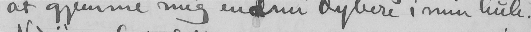at gjemme mig endnu dybere i min hule.
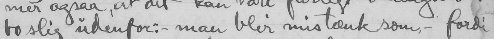bo slig udenfor: - man blir mistænksom, - fordi
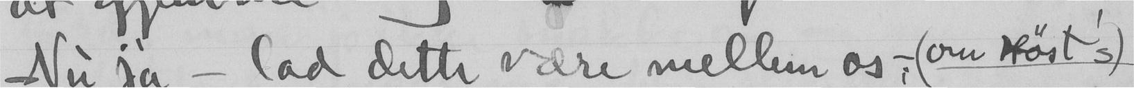Nu ja - lad dette være mellem os - (om Høst's)
